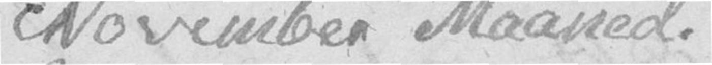November Maaned.
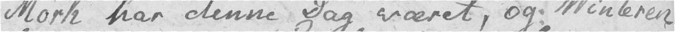Mørk har denne Dag været, og Vinteren
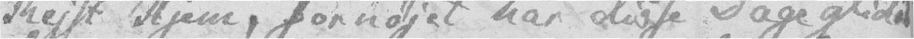Rejst Hjem, fornøjet har disse Dage glidet
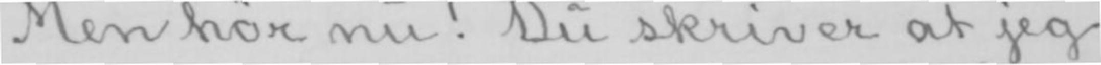Men hør nu! Du skriver at jeg
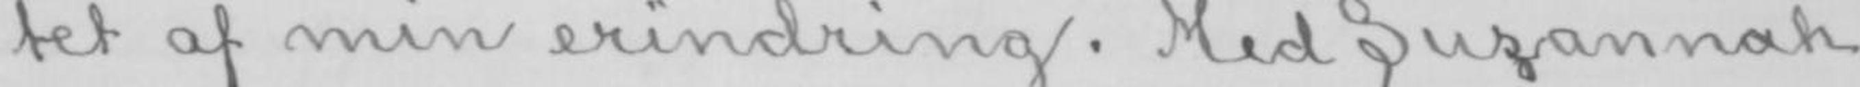tet af min erindring. Med Suszannah
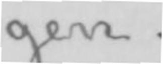gen.
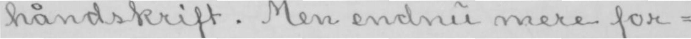håndskrift. Men endnu mere for-
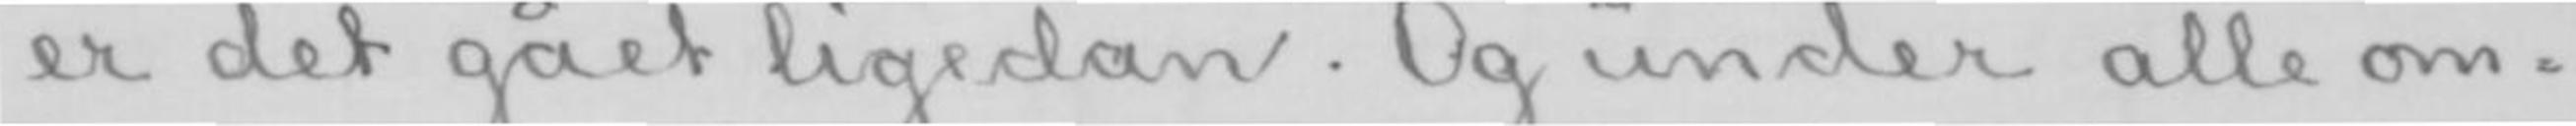er det gået ligedan. Og under alle om-
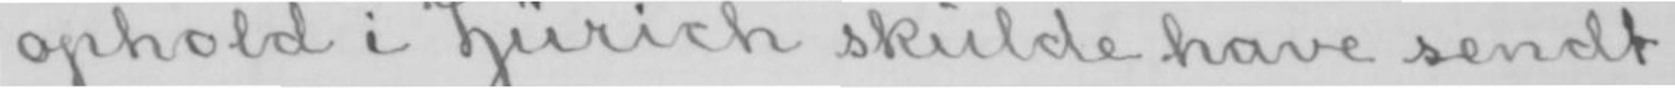ophold i Zürich skulde have sendt
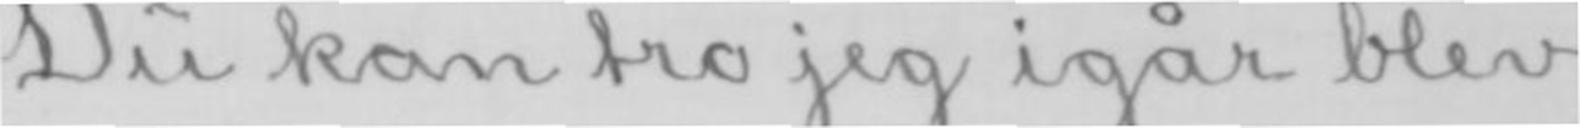Du kan tro jeg igår blev
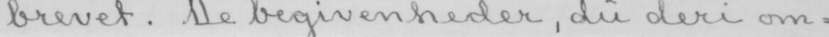brevet. De begivenheder, du deri om-
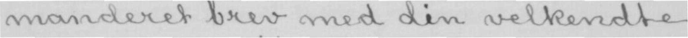manderet brev med din velkendte
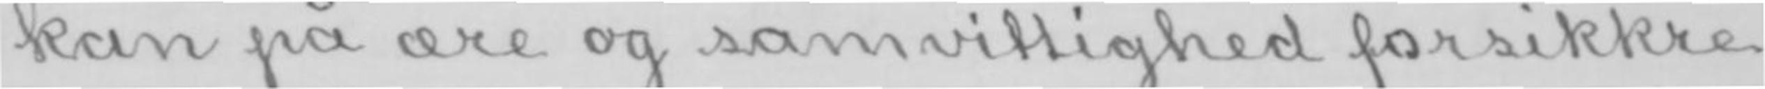kan på ære og samvittighed forsikkre
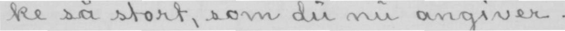ke så stort, som du nu angiver.
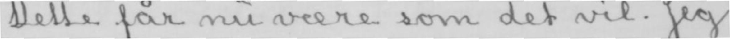Dette får nu være som det vil. Jeg
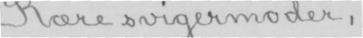Kære svigermoder,
